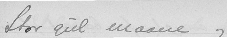Stor gul maane og
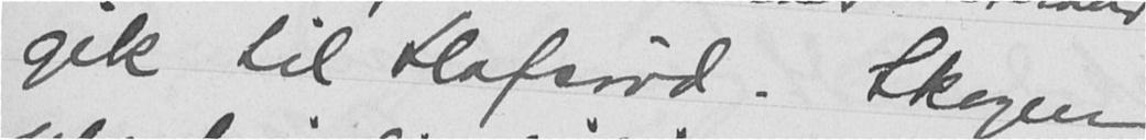gik til Hafsrød. Skogen
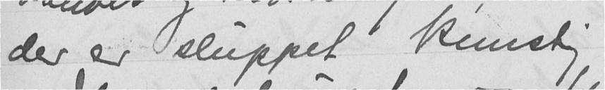der er sluppet kunstig
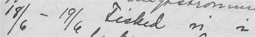18/6 - 19/6 Fisked vi i
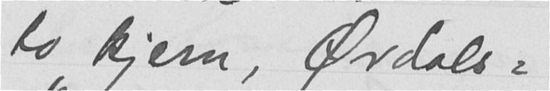to kjern, Ørdals-
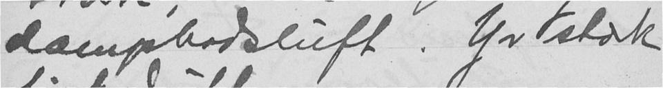dampbaadsluft. Yr stærk
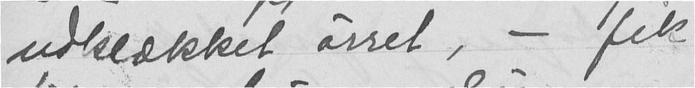udklækket ørret, - fik
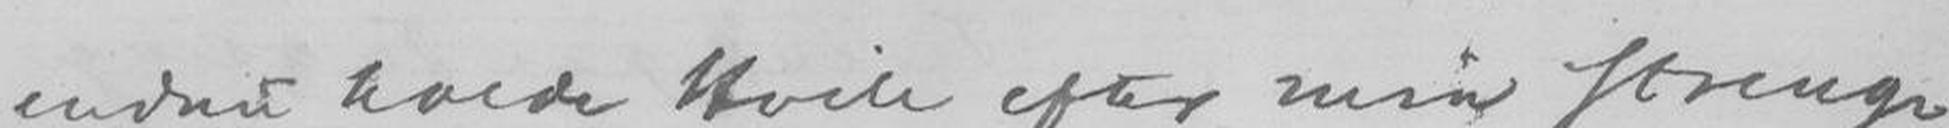endnu holde Hvile efter min strenge
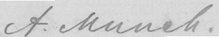A. Munch.
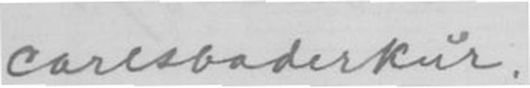Carlsbaderkur.
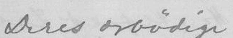Deres ærbødige
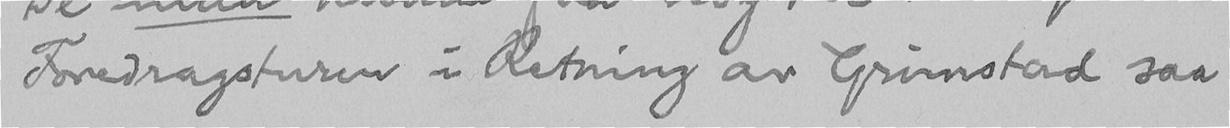Foredragsturen i Retning av Grimstad saa
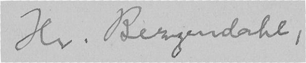Hr. Bergendahl,
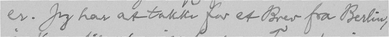er. Jeg har at takke for et Brev fra Berlin,
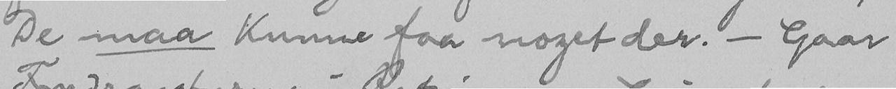De maa kunne faa noget der. - Gaar
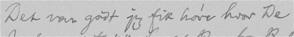Det var godt jeg fik høre hvor De
